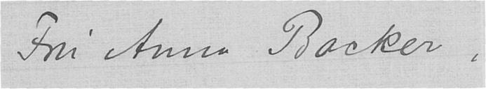Fru Anna Backer,
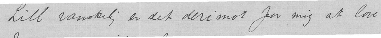Litt vanskelig er det derimot for mig at love
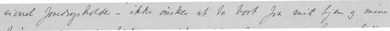sionel foredragsholder – ikke ønsker at ta bort fra mit hjem og mine
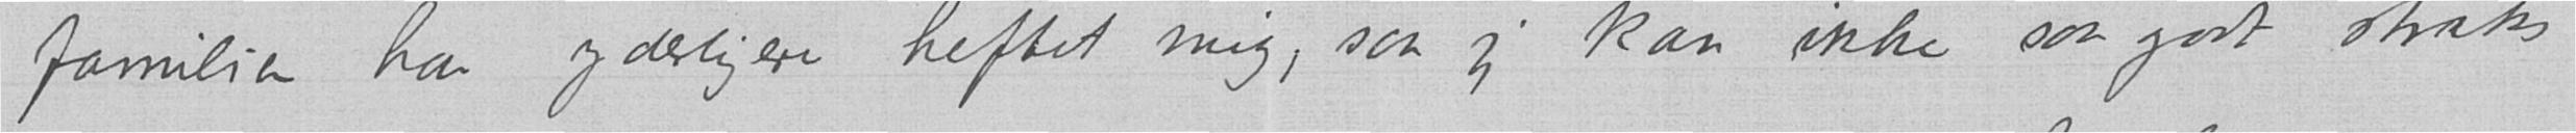familien har yderligere heftet mig, saa jeg kan ikke saa godt straks
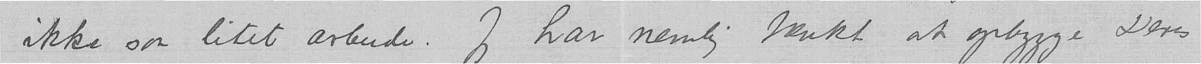ikke saa litet arbeide. Jeg har nemlig tænkt at opbygge Deres
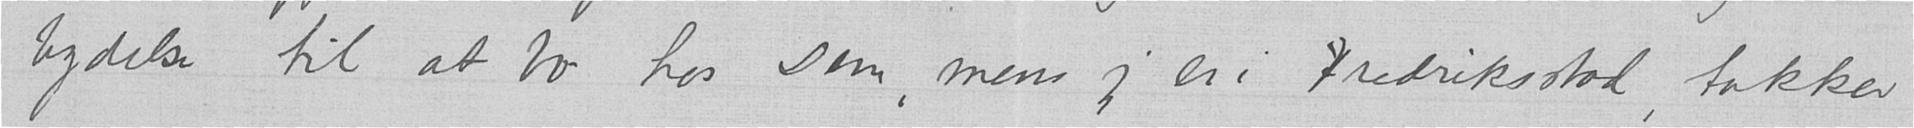bydelse til at bo hos Dem, mens jeg er i Fredriksstad, takker
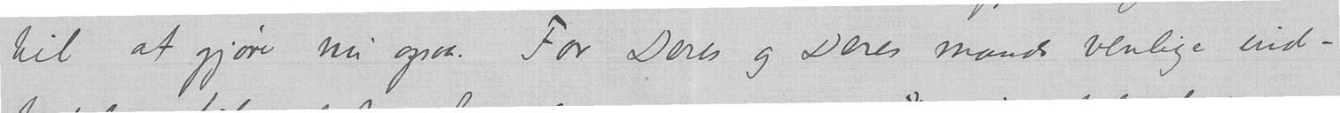til at gjøre nu ogsaa. For Deres og Deres mands venlige ind-
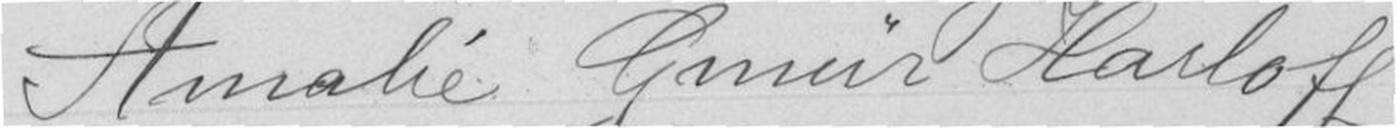Amalie Gmür Harloff.
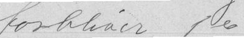forbliver jeg
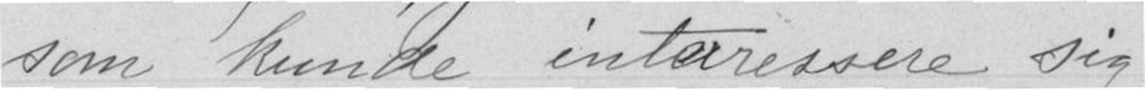som kunde interressere sig
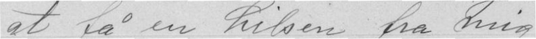at få en hilsen fra mig
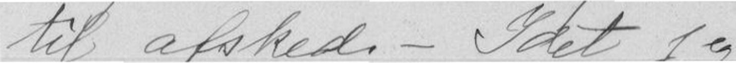til afsked.- Idet jeg
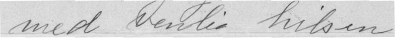med venlig hilsen
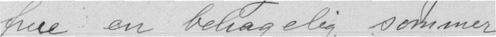frue en behagelig sommer
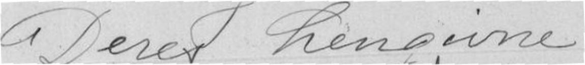Deres hengivne
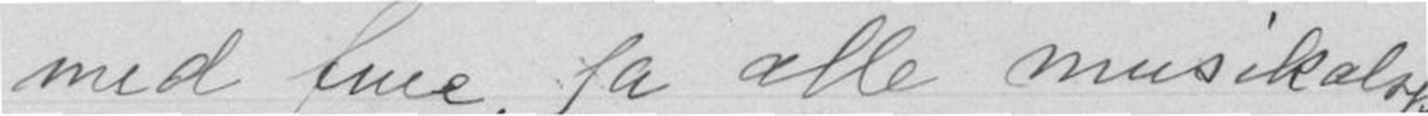med frue, ja alle musikalske
